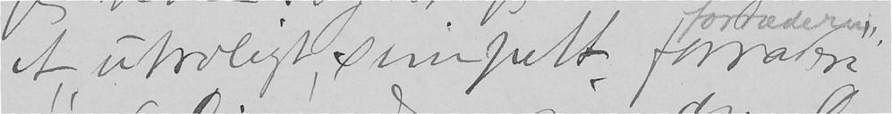et "utroligt, simpelt forræderi"
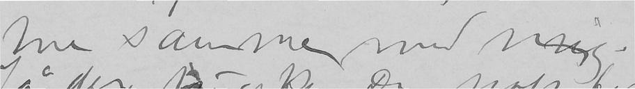nu sammen med mig.
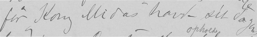før "Kong Midas" havde sét dagens
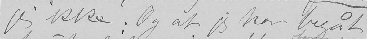jeg ikke. Og at jeg har begåt
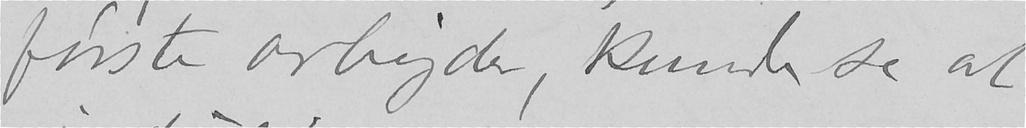første arbejder, kunde se at
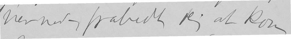hernede frabedt Dig at kom-
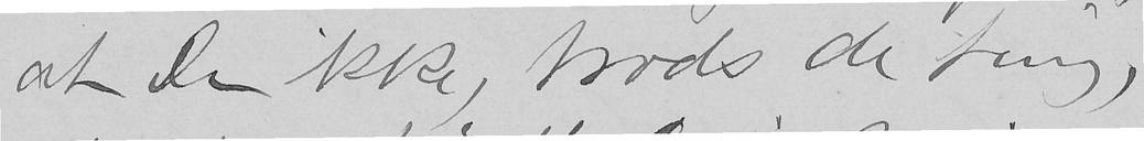at Du ikke, trods de ting,
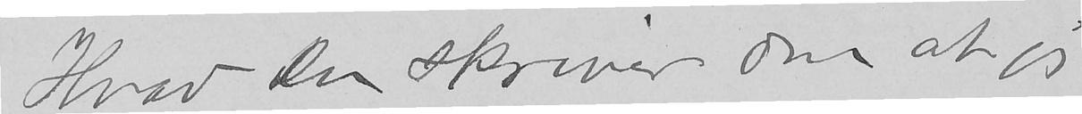Hvad Du skriver om at jeg
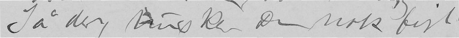Så der, husker Du nok fejl.
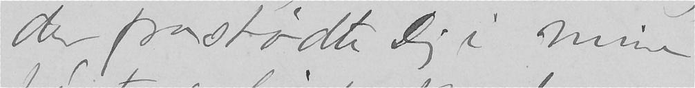der frastødte Dig i mine
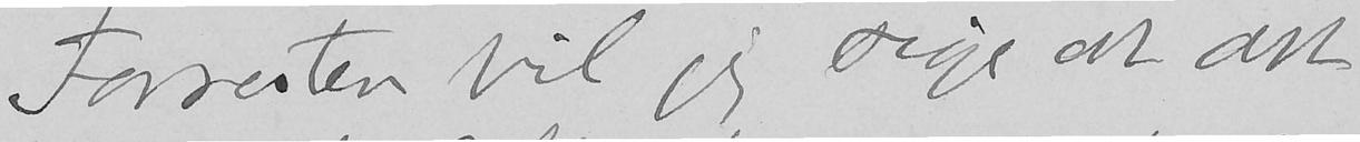Forresten vil jeg sige at det
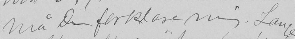må Du forklare mig. Længe
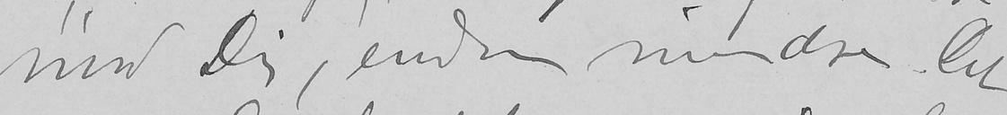mod Dig, endnu mindre. Det
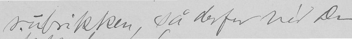rubrikken, så derfor véd Du
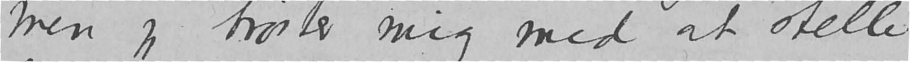Men jeg trøster mig med at stelle
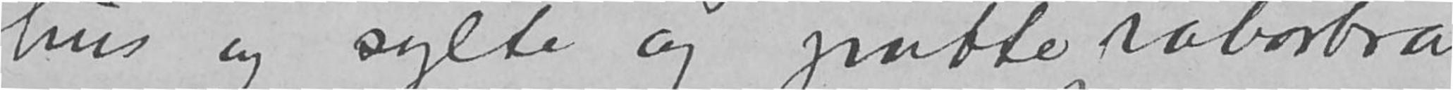hus og sylte og putte rabarbra
